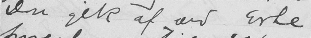Don gik af ved Erte
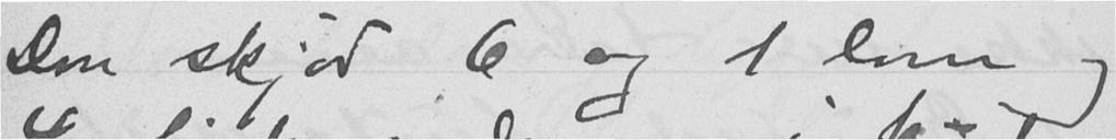Don skjød 6 og 1 lom og
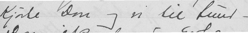Kjørte Don og vi til Sund -
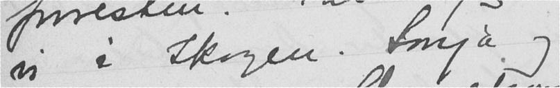vi i Skogen. Sonja og
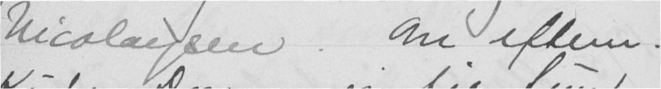Nicolaysen. Om efterm.
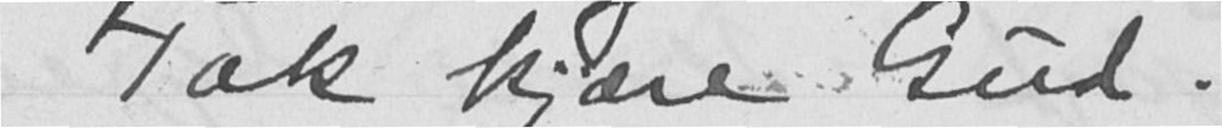Tak kjære Gud.
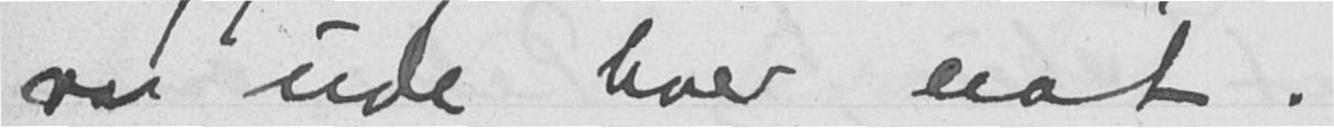var ude hver nat.
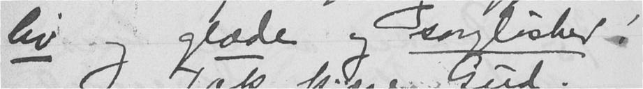liv og glæde og sorgløshed!
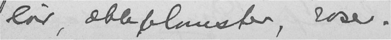løv, æbleblomster, roser.
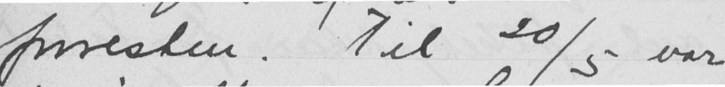forresten. Til 20/5 var
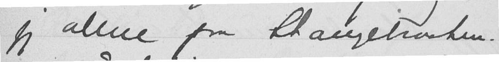jeg alene paa Stangebraaten.
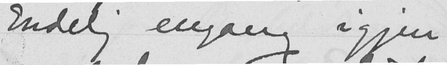Endelig engang igjen
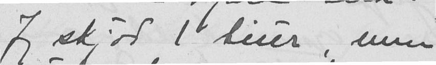Jeg skjød 1 tiur, men
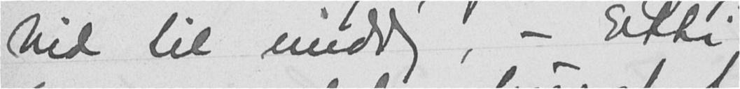hid til middag, - Etti
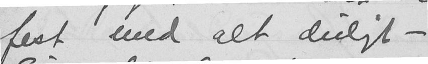fest med alt deiligt -
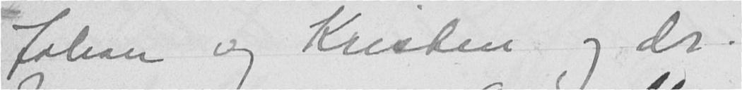Johan og Kristen og dr.
